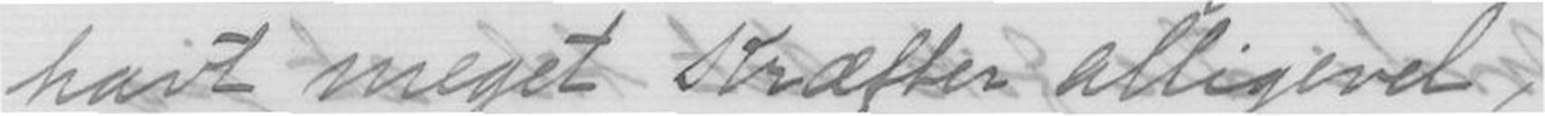havt meget Kræfter alligevel,
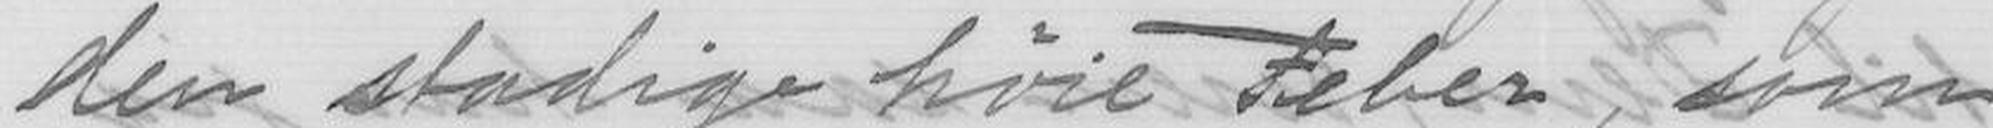den stadige høie Feber, som
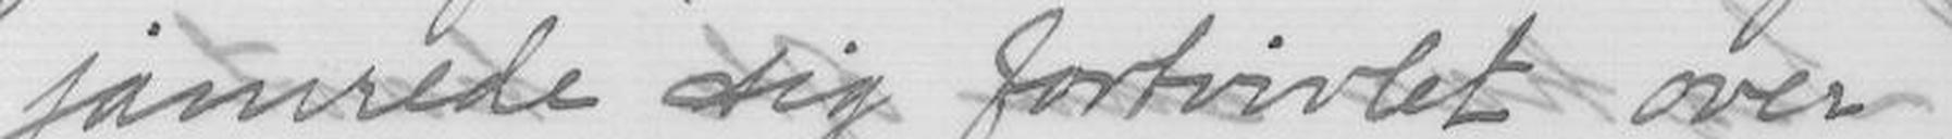jamrede dig fortvilet over
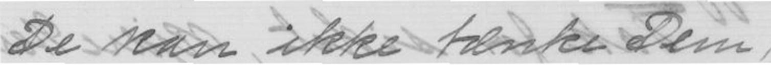De man ikke tænker Dem
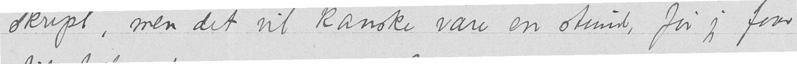skript, men det vil kanske vare en stund, før jeg faar
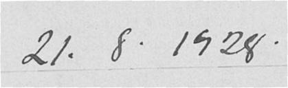21.8.1928
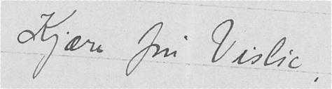Kjære fru Vislie,
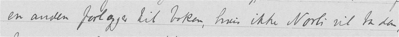en anden forlægger til boken, hvis ikke Norli vil ta den,
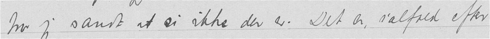tror jeg sandt at si ikke der er. Det er ialfald efter
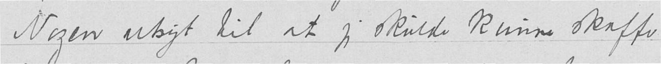Nogen utsigt til at jeg skulde kunne skaffe
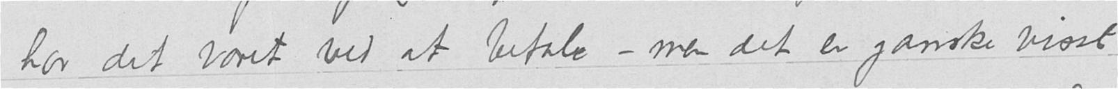har det været ved at betale – men det er ganske visst
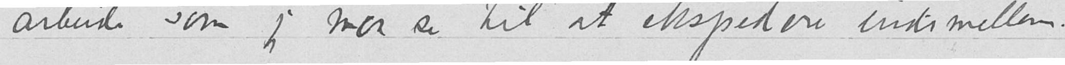arbeide som jeg maa se til at ekspedere indimellem.
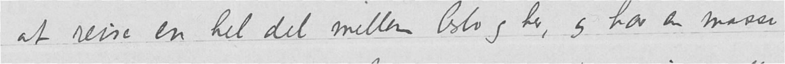at reise en hel del mellem Oslo og her, og har en masse
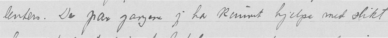lenters. De par gangene jeg har kunnet hjelpe med slikt
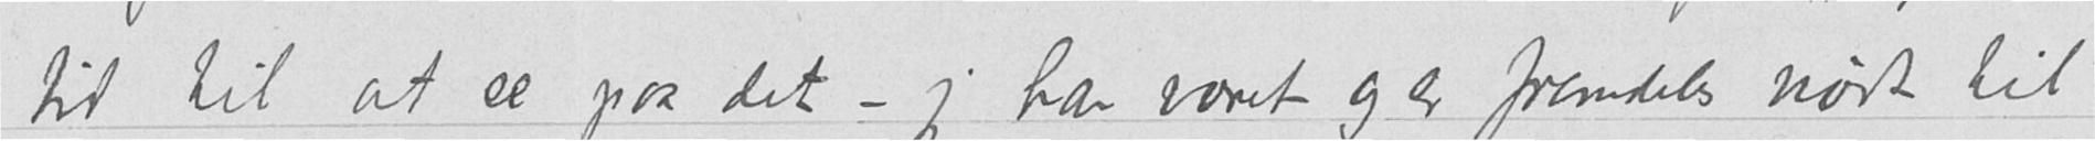tid til at se paa det – jeg har været og er fremdels nødt til
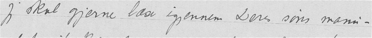jeg skal gjerne læse igjennem Deres søns manu-
